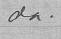da.
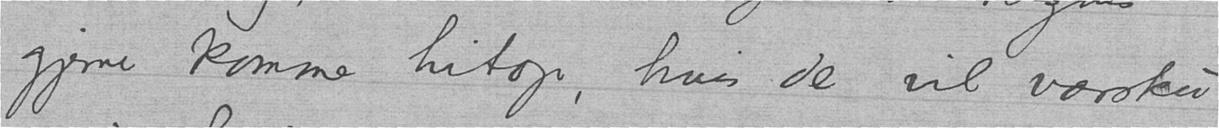gjerne komme hitop, hvis De vil varsku
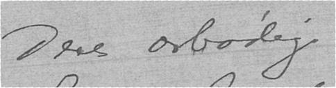Deres ærbødige
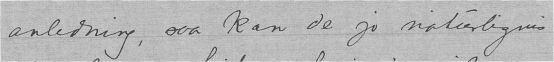anledning, saa kan De jo naturligvis
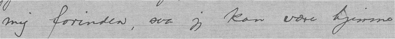mig forinden, saa jeg kan være hjemme
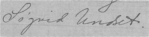Sigrid Undset.
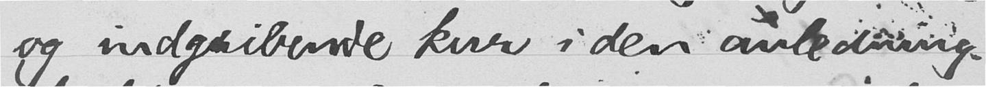og indgribende kur i den anledning.
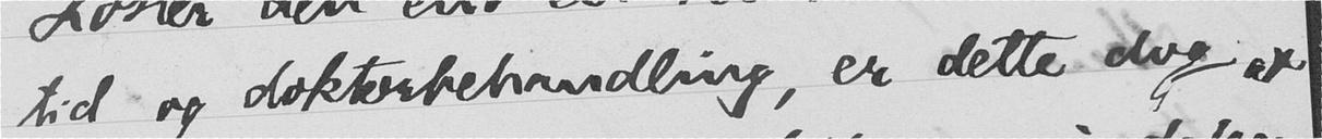tid og doktorbehandling, er dette dog at
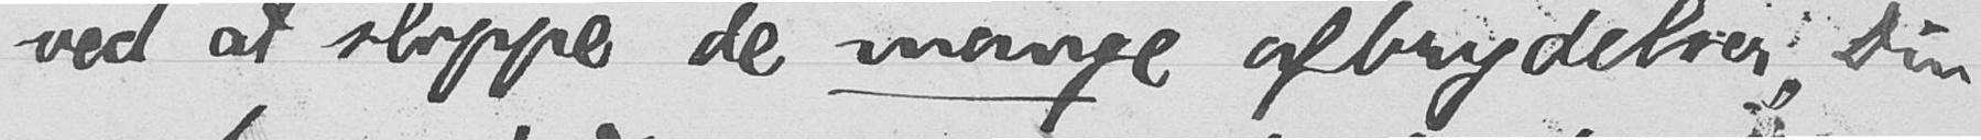ved at slippe de mange afbrydelser Din
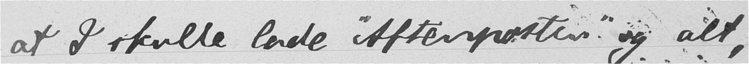at I skulle lade "Aftenposten" og alt,
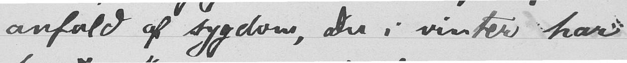anfald af sygdom, Du i vinter har
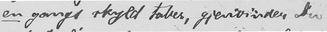en gangs skyld taber, gjenvinder Du
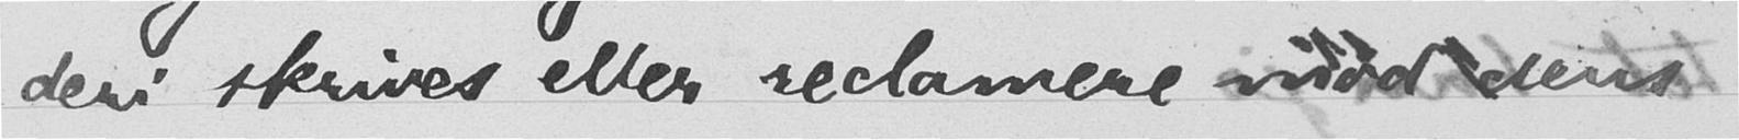deri skrives eller reclamere mod dens
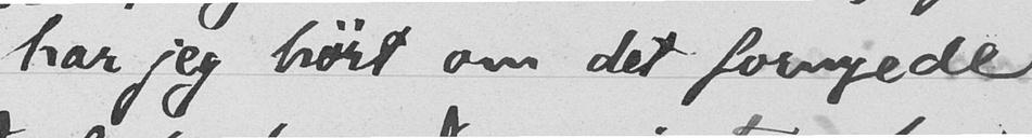har jeg hørt om det fornyede
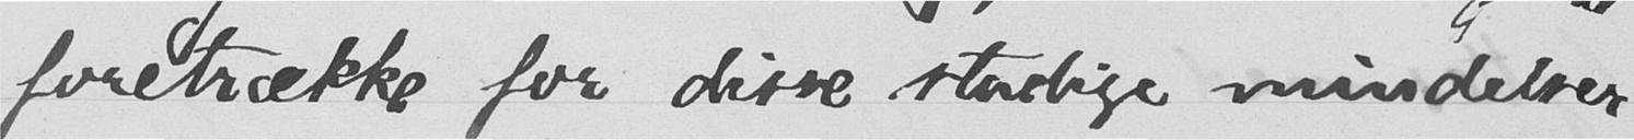foretrække for disse stadige mindelser
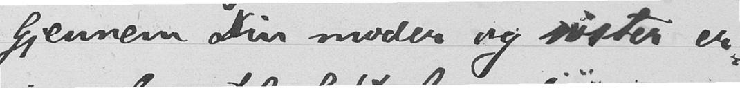Gjennem Din moder og søster er-
